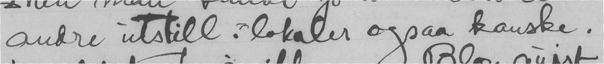andre utstill.- lokaler ogsaa kanske.
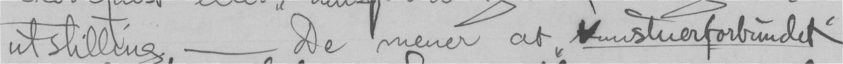utstilling - De mener at "Kustnerforbundet"
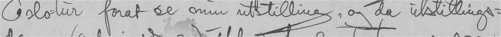Oslo-tur forat se min utstilling, og da utstillings-
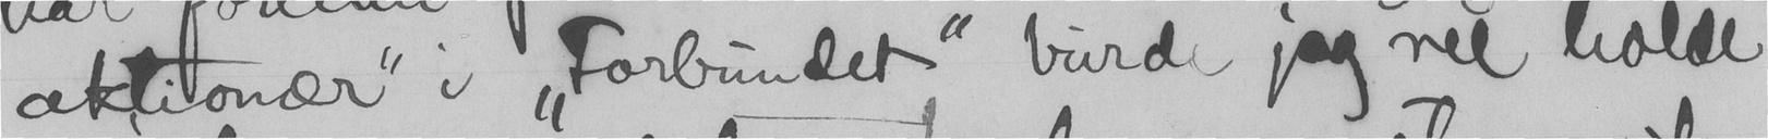"aktionær" i "Forbundet" burde jeg vel holde
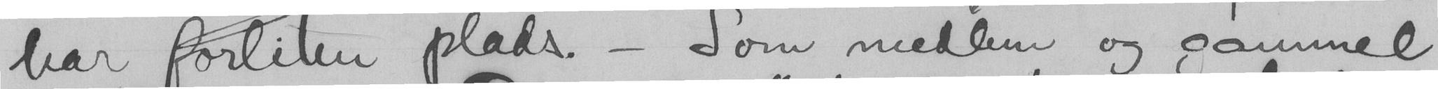har forliten plads. - Som medlem og gammel
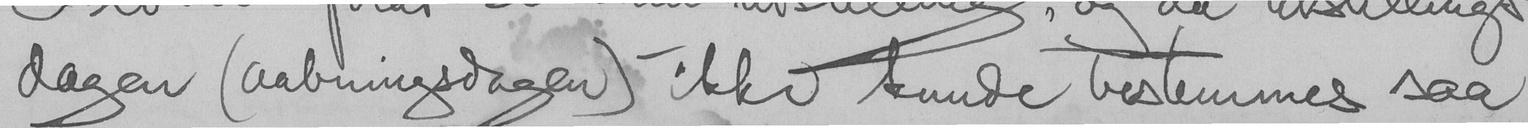dagen (aabningedagen) ikke kunde bestemmes saa
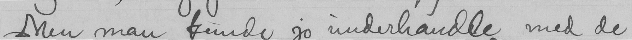Men man kunde jo underhandle med de
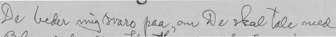De beder mig svare paa om De skal tale med
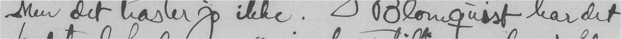Men det haster jo ikke. Blomquist har det
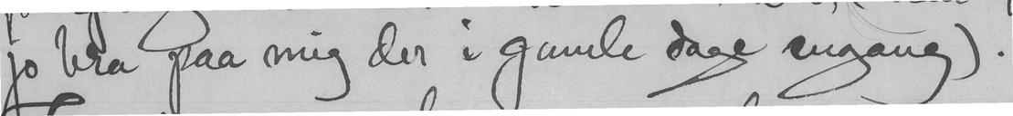jo bra paa mig der i gamle dage engang).
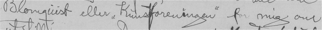Blomquist eller "Kunsforeningen" for mig om
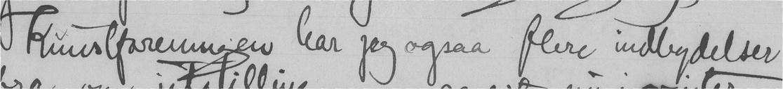Kunstforeningen har jeg ogsaa flere indbydelser
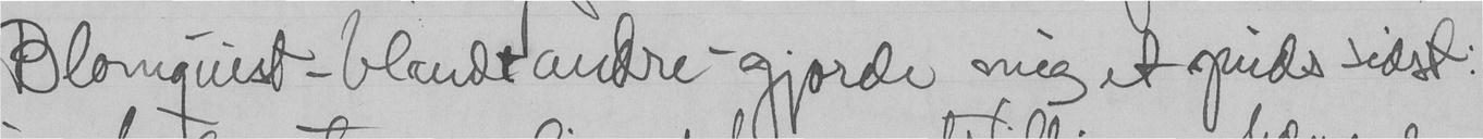Blomquist- blandt andre - gjorde mig et puds sidst:
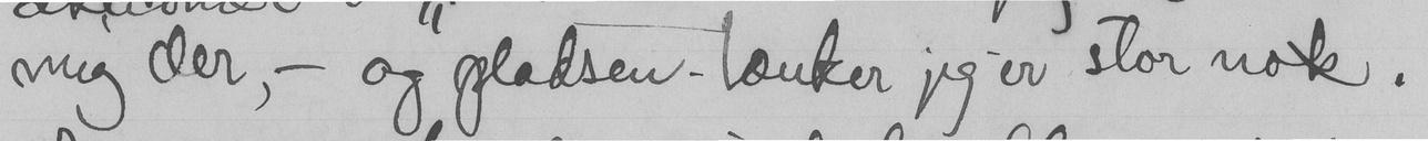mig der, - og pladsen - tænker jeg er stor nok.
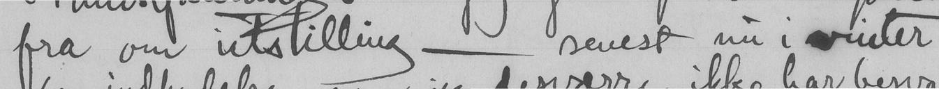fra om utstilling - senest nu i vinter
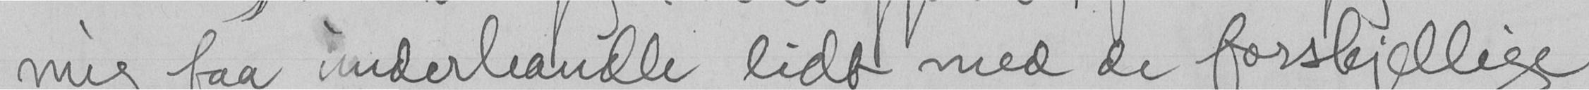mig faa underhandle lidt med de forskjellige
